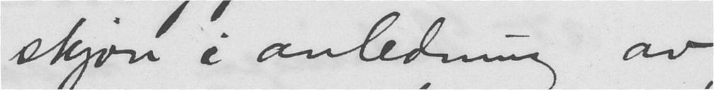skjøn i anledning av
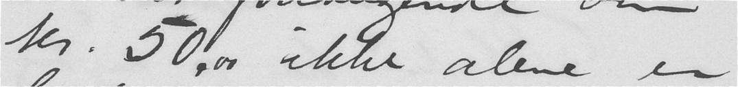kr. 50,00 ikke alene er
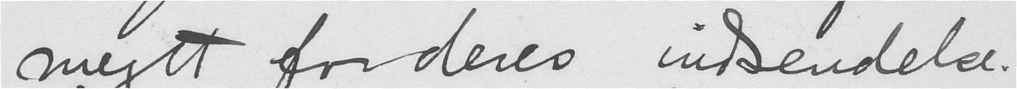meget for deres indsendelse.
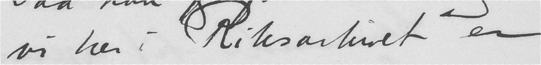vi her i Riksarkivet er
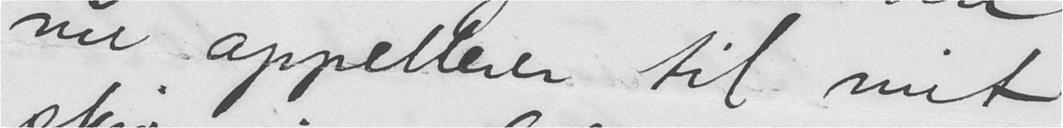nu appellerer til mit
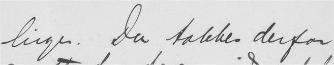linger. Du takkes derfor
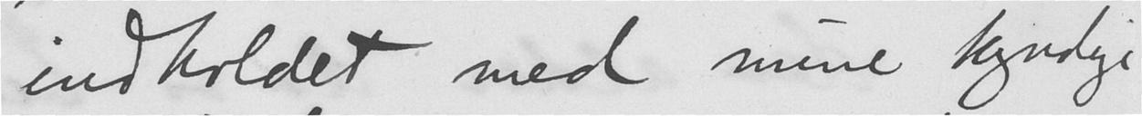indholdet med mine kyndige
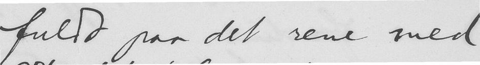fuldt paa det rene med
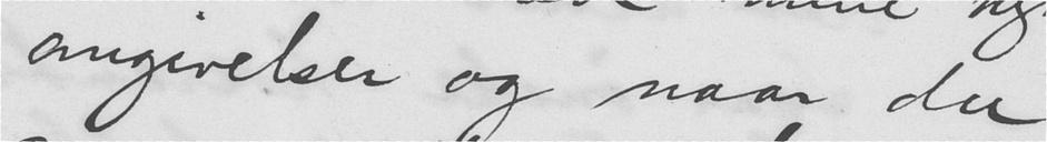omgivelser og naar du
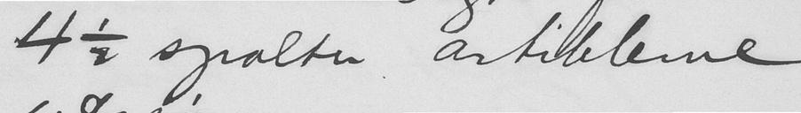4 ½ spalter artiklerne
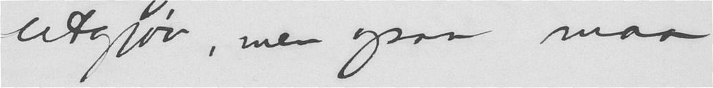utgjør, men ogsaa maa
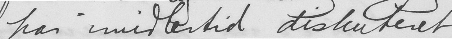har imidlertid diskuteret
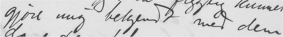gjøre mig bekjendt med dem
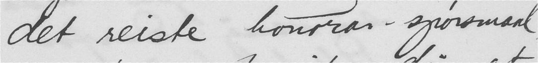det reiste honorar-spørsmaal,
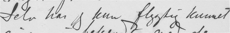Selv har jeg kun flygtig kunnet
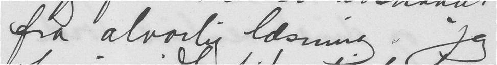fra alvorlig læsning. Jeg
